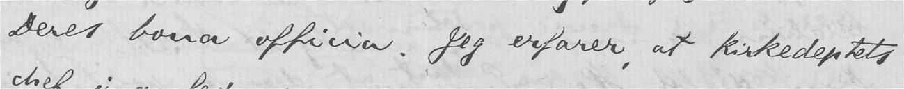Deres bona officia. Jeg erfarer, at kirkedeptets
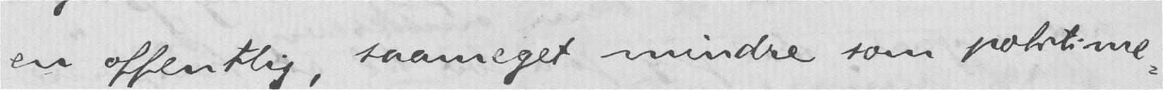en offentlig, saameget mindre som politime-
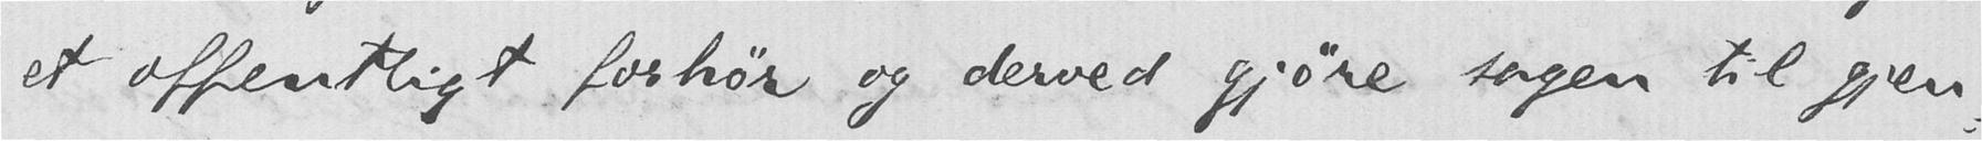et offentligt forhør og derved gjøre sagen til gjen-
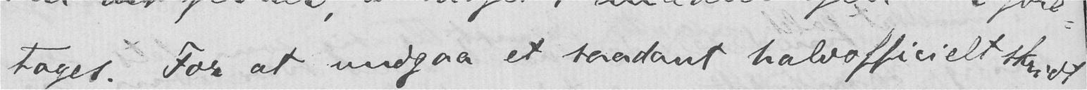tages. For at undgaa et saadant halvofficielt skridt,
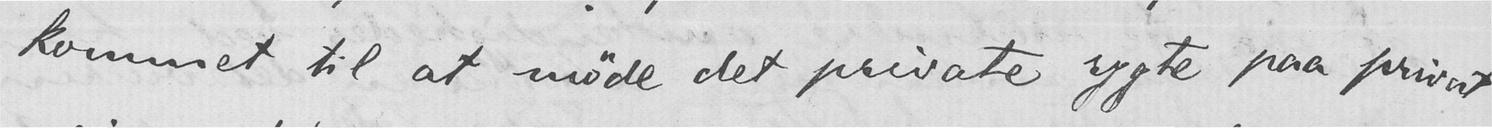kommet til at møde det private rygte paa privat
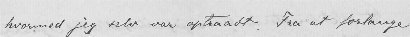hvormed jeg selv var optraadt. Fra at forlange
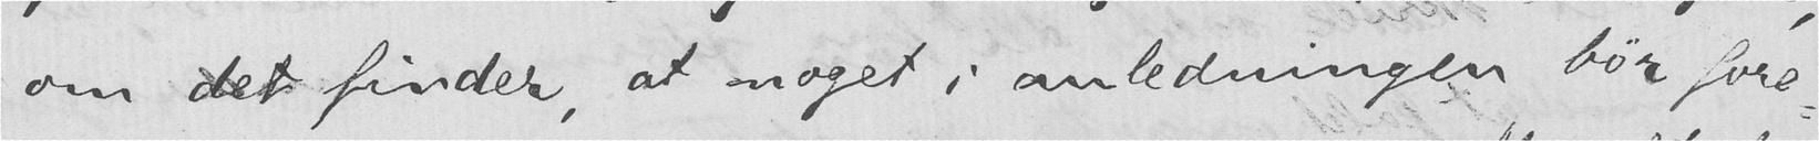om det finder, at noget i anledningen bør fore-
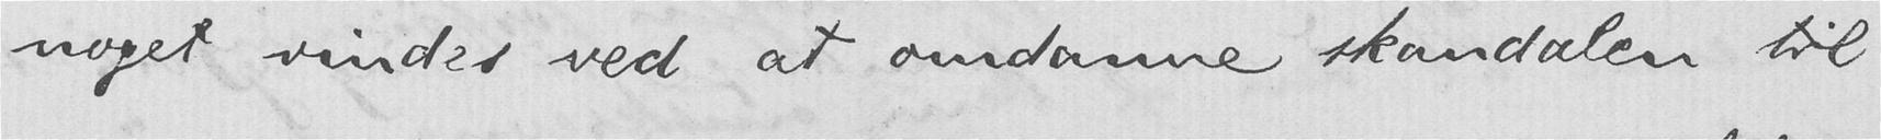noget vindes ved at omdanne skandalen til
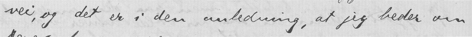vei, og det er i den anledning, at jeg beder om
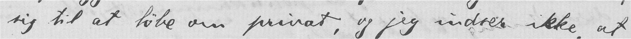sig til at løbe om privat, og jeg indser ikke, at
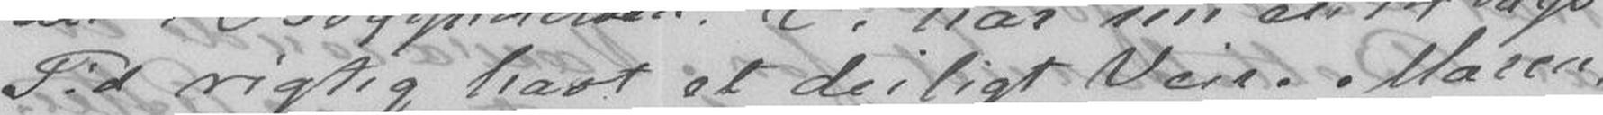Tid rigtig havt et deiligt Veir. Maren
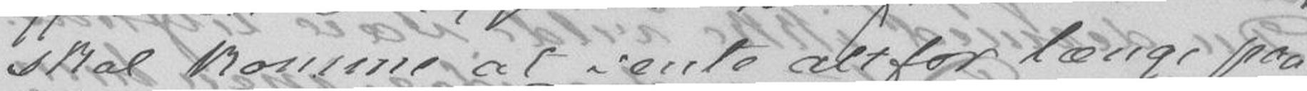skal komme at vente altfor længe paa
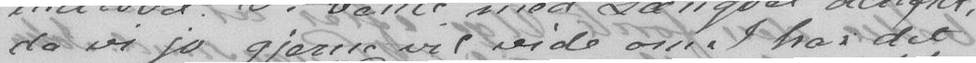da vi jo gjerne vil vide om I har det
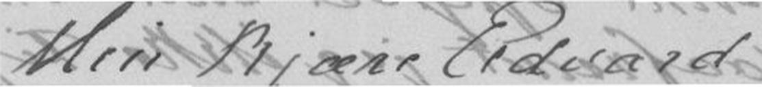Min kjære Edvard
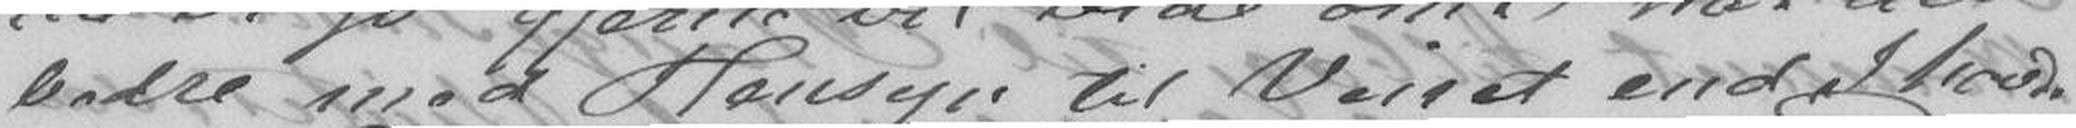bedre med Hensyn til Veiret end I havde
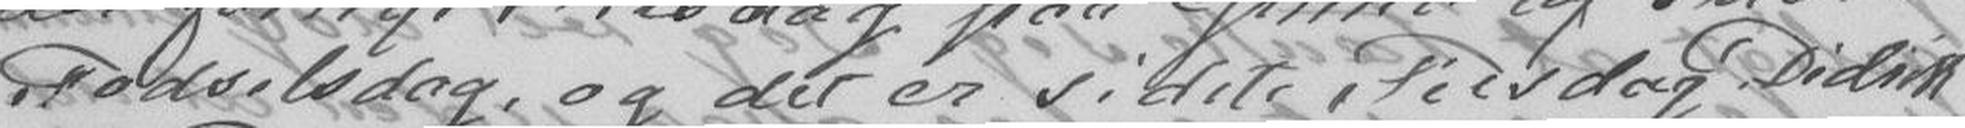Fødselsdag, og det er sidste Tirsdag Didrik
